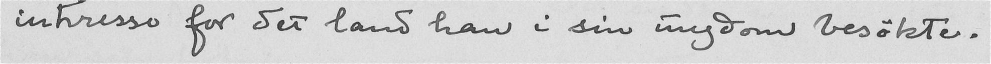interesse for det land han i sin ungdom besøkte.
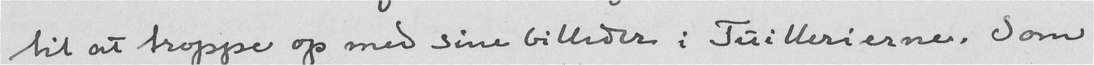til at troppe op med sine billeder i Tuillerierne. Som
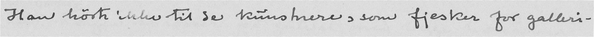Han hørte ikke til de kunstnere, som fjesker for galleri-
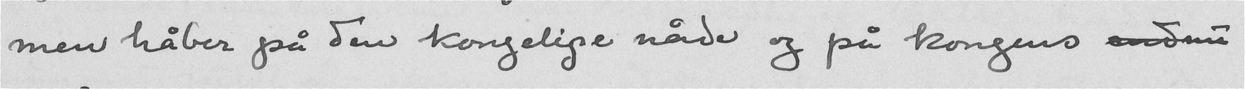men håber på den kongelige nåde og på kongens endnu
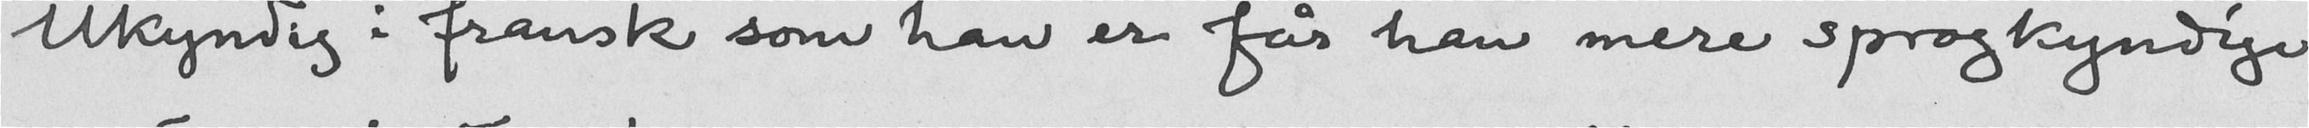Ukyndig i fransk som han er får han mere sprogkyndige
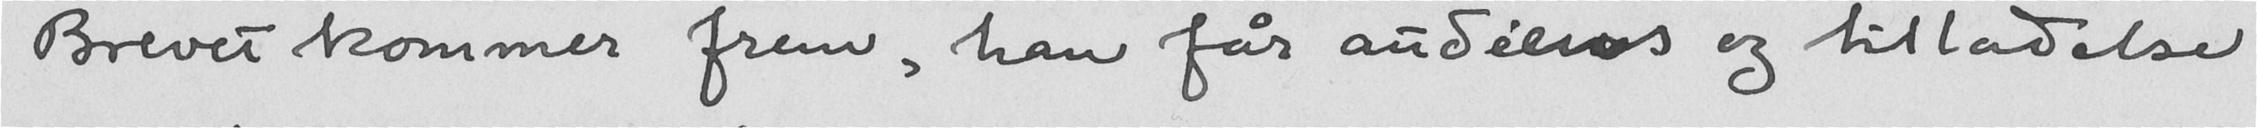Brevet kommer frem, han får audiens og tilladelse
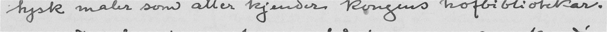tysk maler som atter kjender kongens hofbibliotekar.
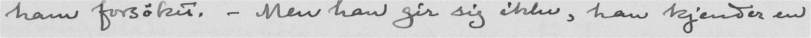ham forsøket. - Men han gir sig ikke, han kjender en
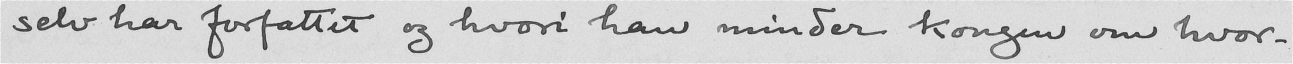selv har forfattet og hvori han minder kongen om hvor-
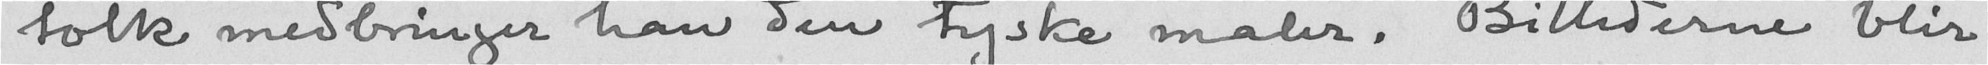tolk medbringer han den tyske maler. Billederne blir
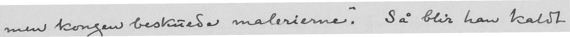men kongen beskuede malerierne". Så blir han kaldt
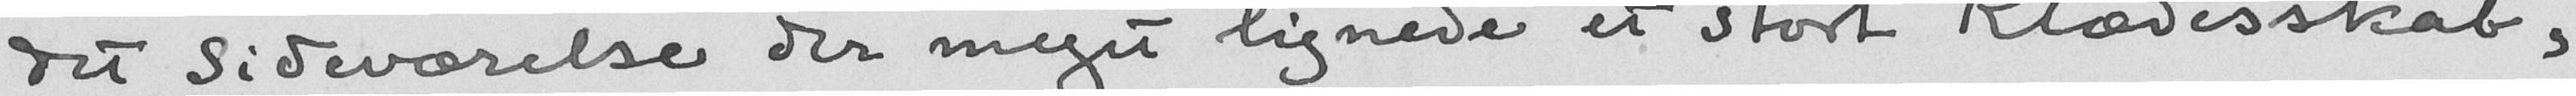det sideværelse der meget lignede et stort Klædesskab,
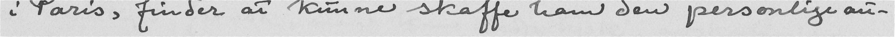i Paris, finder at kunne skaffe ham den personlige au-
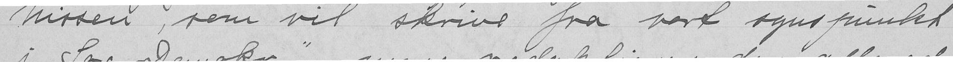Nissen, som vil skrive fra vort synspunkt
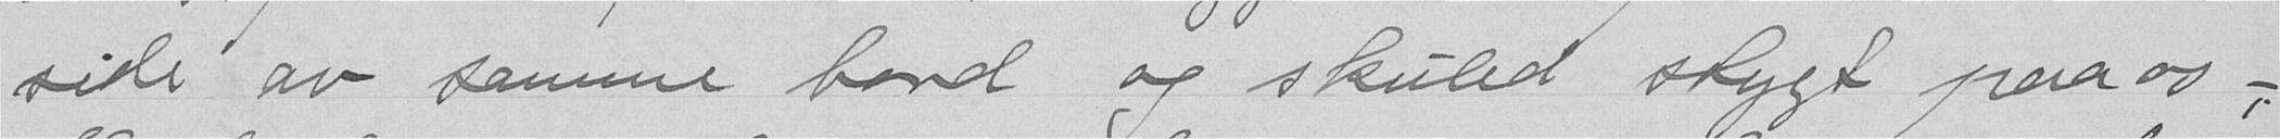side av samme bord og skuled skygt paa os, -
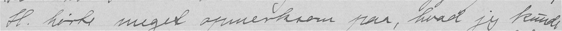H. hørte meget opmerksom paa, hvad jeg kunde
